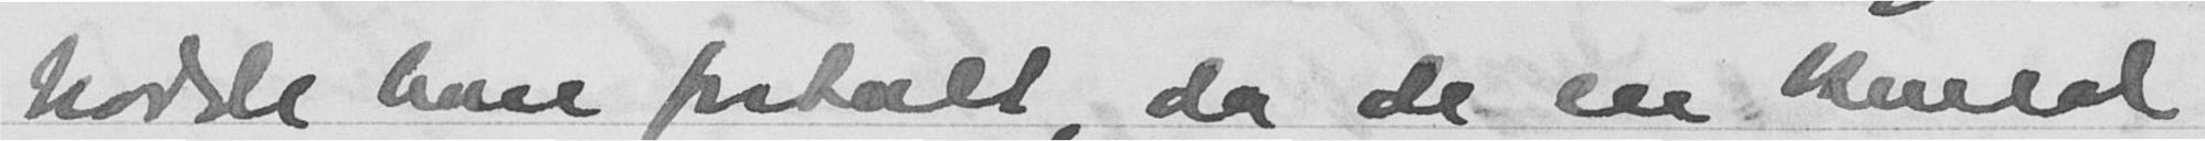hadde han fortalt, da de en kveld
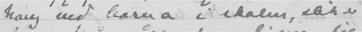hang ved barna i skolen, slik er
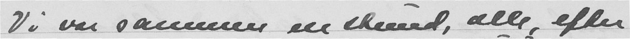Vi var sammen en stund, alle efter
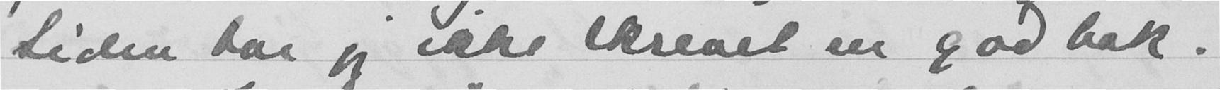Siden har jeg ikke skrevet en god bok.
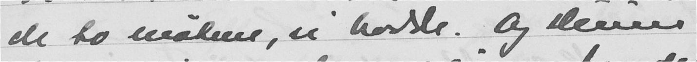de to møtene, vi hadde. Og Duun
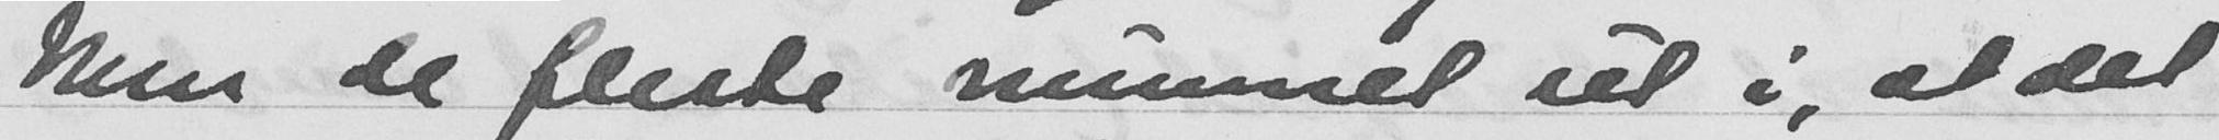Men de fleste munnet ut i, at det
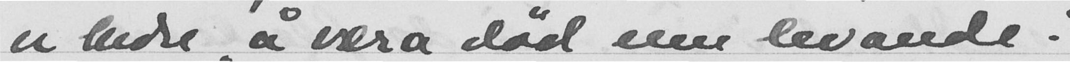er bedre "å være død en levende."
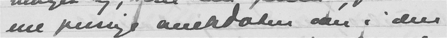ene pussige anekdoten over i den
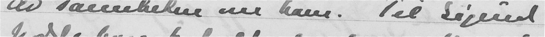av sannheten om ham. Til Sigurd
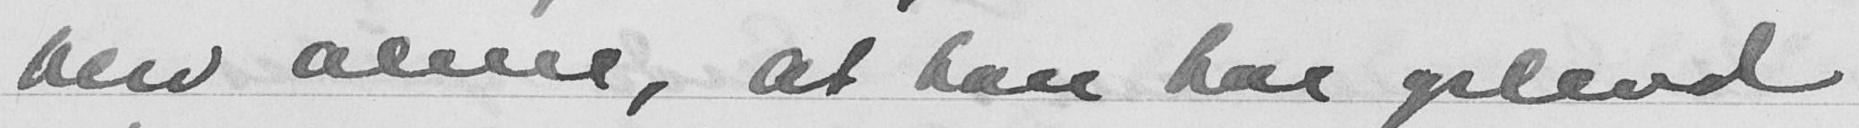blev alene, at han har oplevd
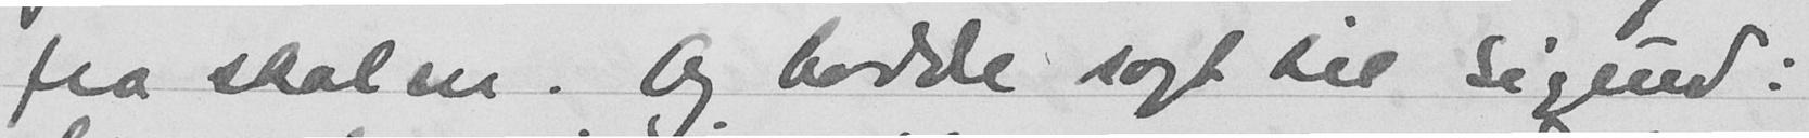fra skolen. Og hadde sagt til Sigurd:
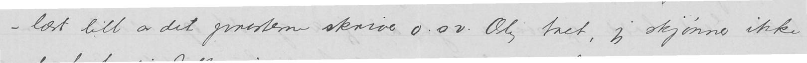–læst litt av det presterne skriver o.sv. Ærlig talt, jeg skjønner ikke
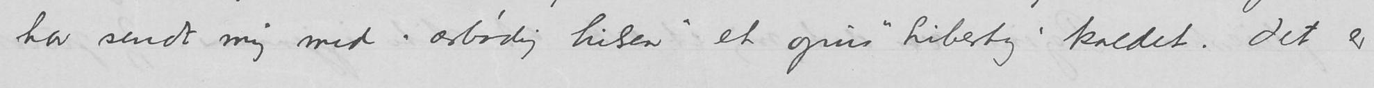har sendt mig med «ærbødig hilsen» et opus «Liberty» kaldet. Det er
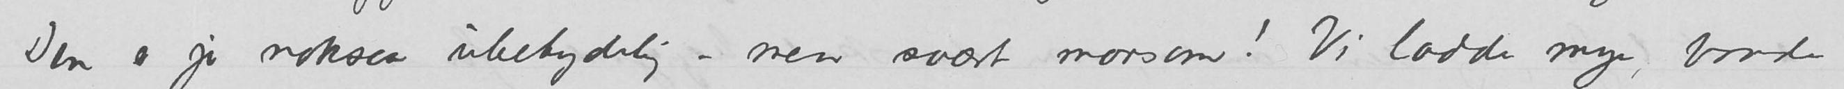Den er jo noksaa ubetydelig – men svært morsom! Vi lodde mye, baade
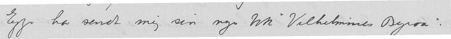Egge har sendt mig sin nye bok «Vilhelmines Byraa».
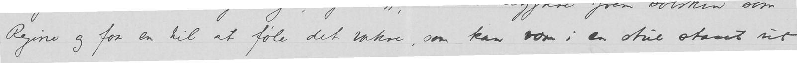Regine og faa en til at føle det vakre, som kan være i en stue staset ut
